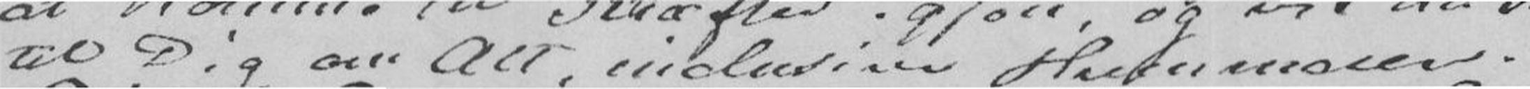til Dig om Alt, inclusive Hummeren.
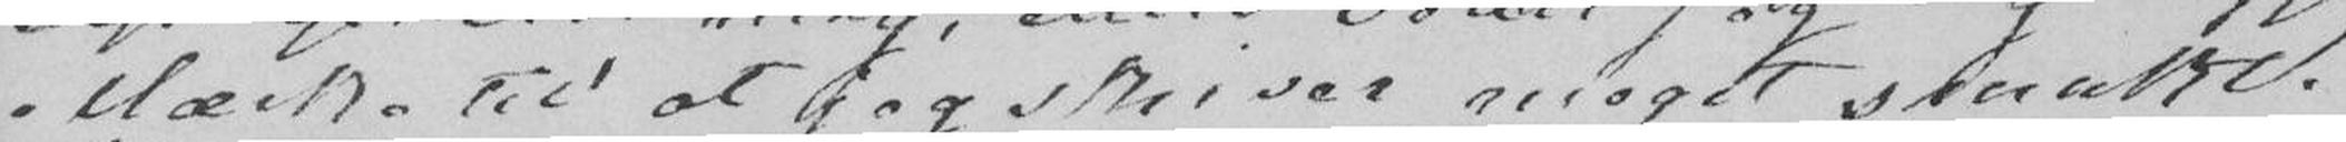Mærke til at jeg skriver meget smukt.
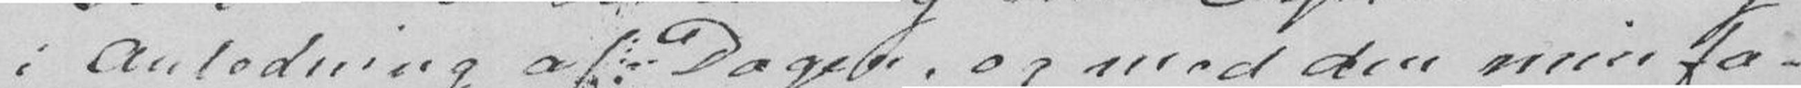i Anledning af Dagen, og med den min fa-
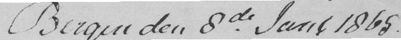Bergen den 8de Juni 1865
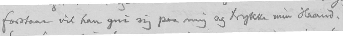forstaar vil han gni sig paa mig og trykke min Haand.
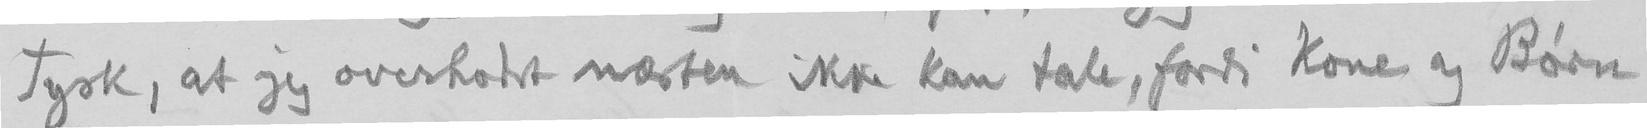Tysk, at jeg overhodet næsten ikke kan tale, fordi Kone og Børn
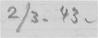2/3. 43.
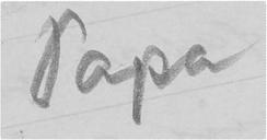Papa
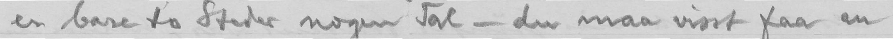er bare to Steder nogen Tal - du maa visst faa en
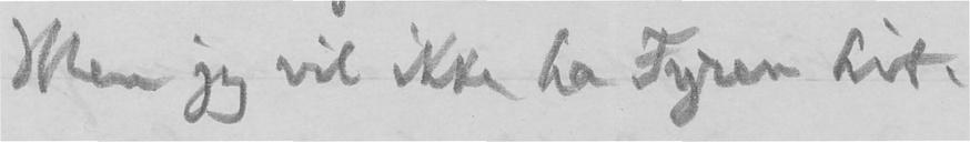Men jeg vil ikke ha Fyren hit.
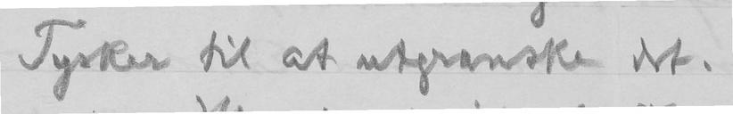Tysker til at utgranske det.
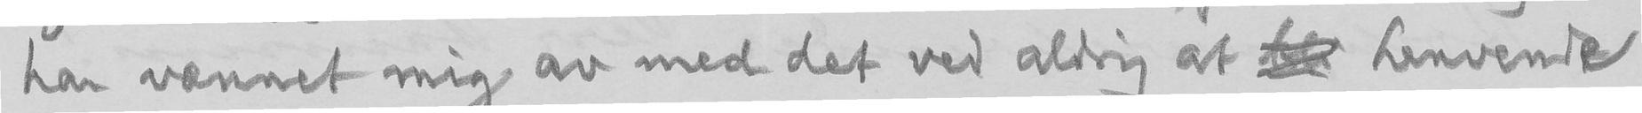har vænnet mig av med det ved aldrig at bla henvende
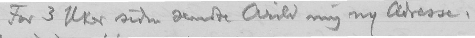For 3 Uker siden sendte Arild mig ny Adresse.
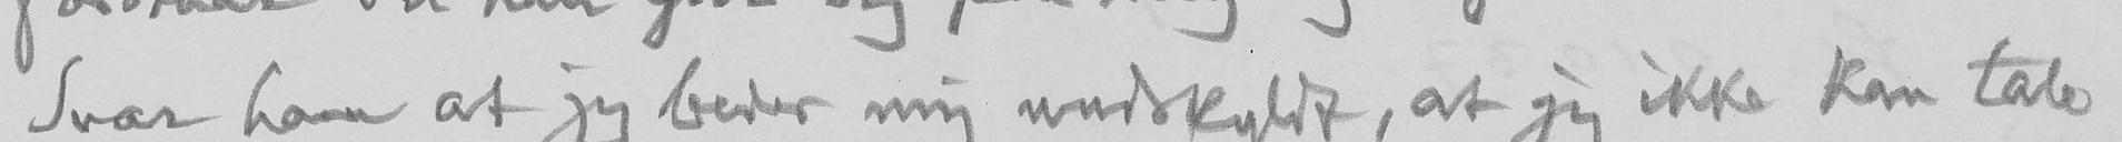Svar ham at jeg beder mig undskyldt, at jeg ikke kan tale
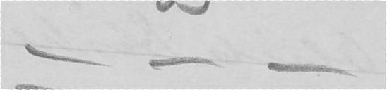- - -
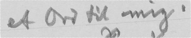et Ord til mig.
